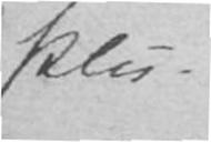Plu-
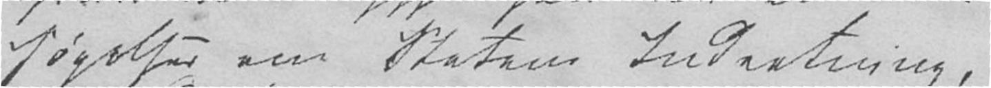søgelser om Statens Indretning,
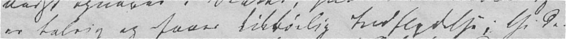er talrig og faaer tilbørlig Indflydelse; thi der
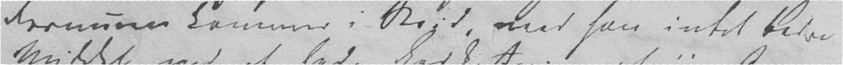Formuen kommer i Strid, veed han intet bedre
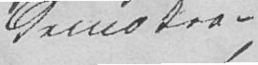demokra-
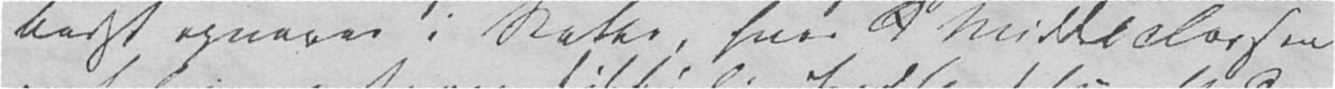bedst opnaaes i Stater, hvor d Middelclassen
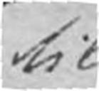til-
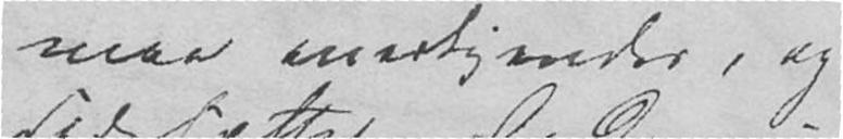maa erkjendes, og
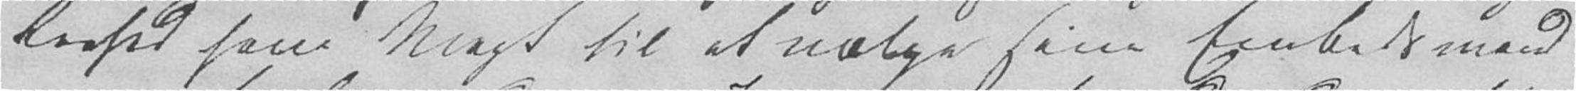Raahed have Magt til at vælge sine Embedsmænd
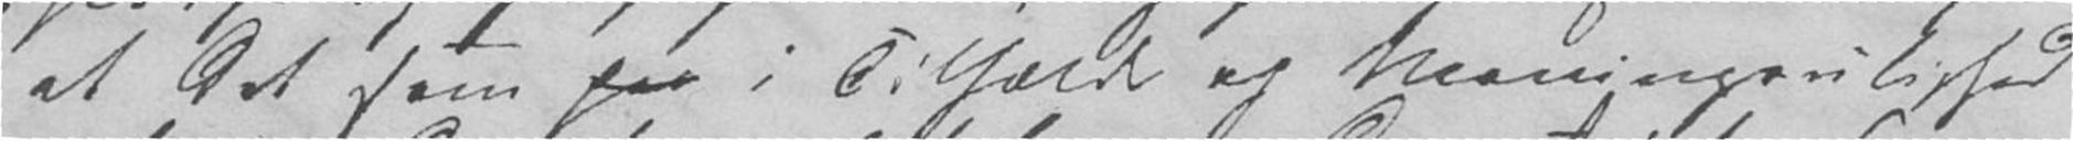at det som paa i Tilfælde af Meningsulighed
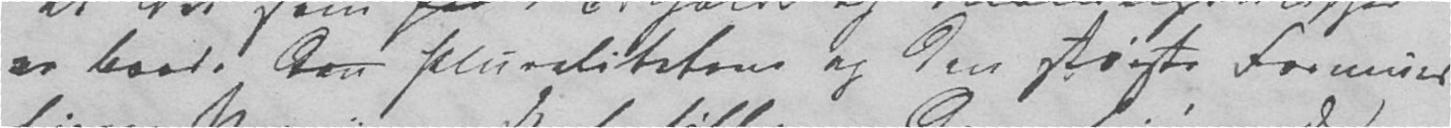er baade den Pluralitetens og den største Formues
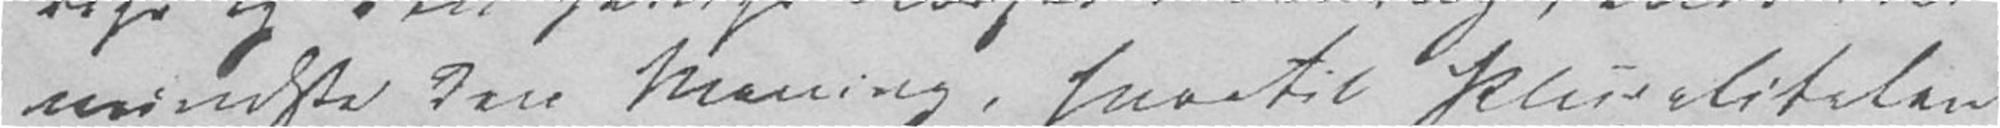mindste den Mening, hvortil Pluraliteten
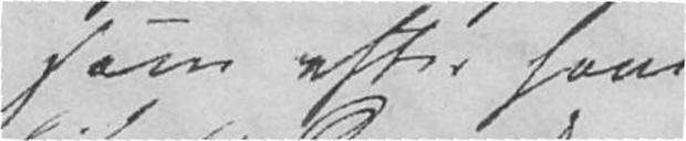som efter hans
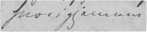hvorigjennem
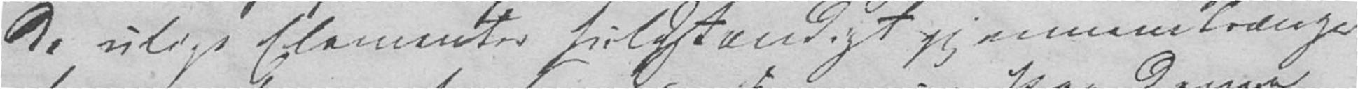de ulige Elementer fuldstændigt gjennemtrænge
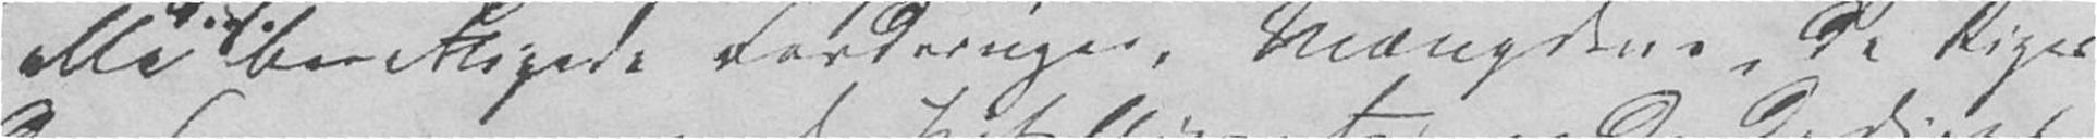alle berettigede Fordringer, Mængdens, de Riges
